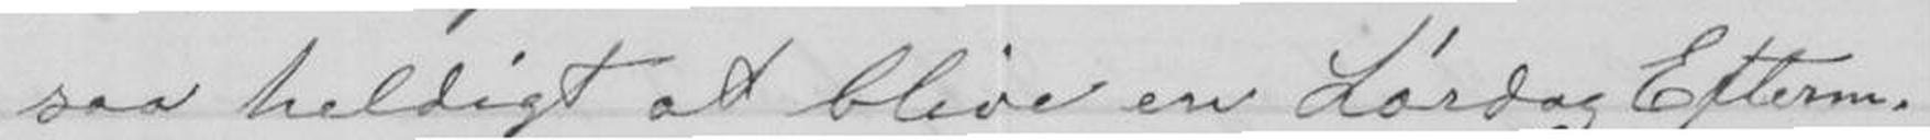saa heldigt at blive en Lørdag Efterm.
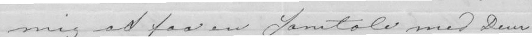mig at faa en Samtale med Dem
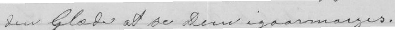den Glæde at se Dem igaarmorges.
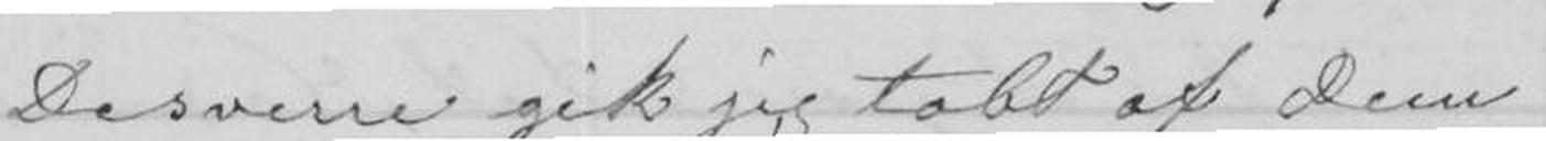Desverre gik jeg tabt af dem
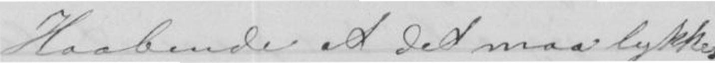Haabende at det maa lykkes
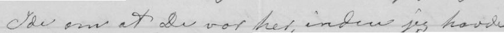Ide om at Du var her, inden jeg havde
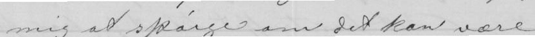mig at spørge om det kan være
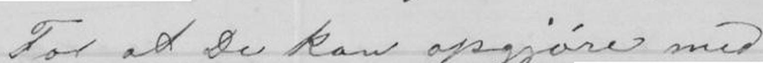For at Du kan opgjøre med
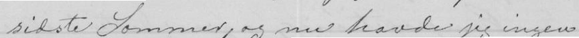sidste Sommer, og nu havde jeg ingen
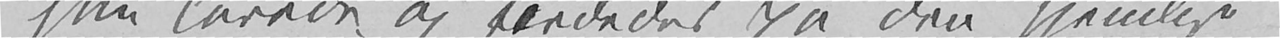hun levede og færdedes på den hjemlige
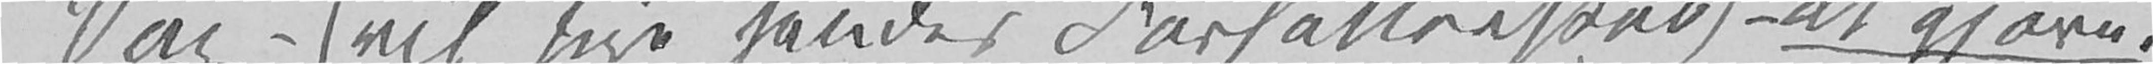Sag – (vil sige hendes Forfatterskab) – at gjøre.»
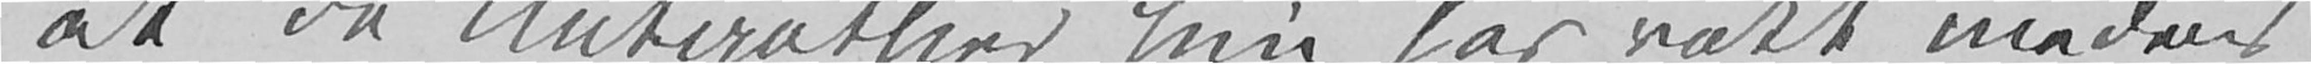at «de Antipathier hun har vakt medens
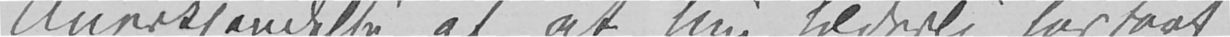Anerkjendelse af at hun hæderlig har ført
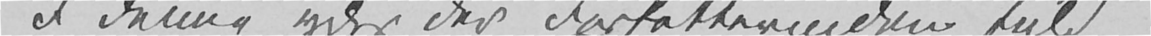I denne ydes der Forfatterinden fuld
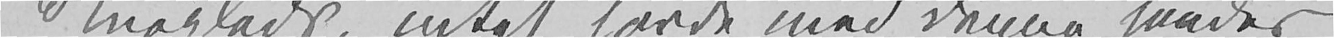Skueplads, intet havde med denne hendes
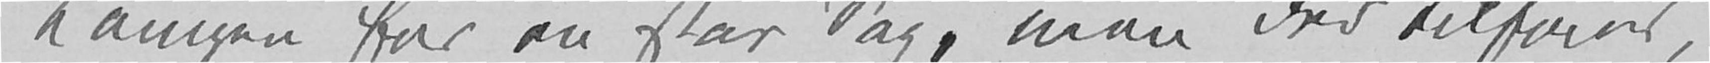Kampen for en stor Sag, men der tilføies,
